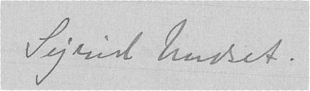Sigrid Undset.
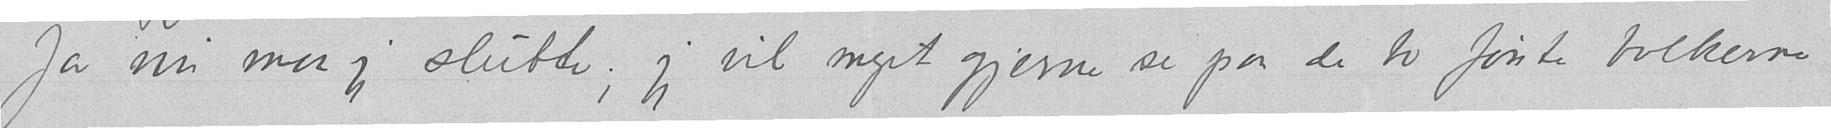Ja nu maa jeg slutte; jeg vil meget gjerne se paa de to første bolkerne
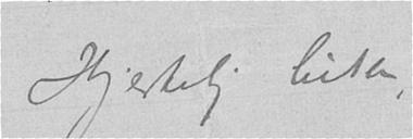Hjertelig hilsen,
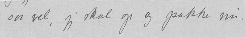saa vel, jeg skal op og pakke nu.
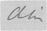din
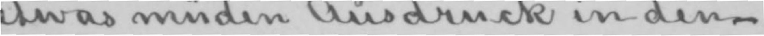etwas müden Ausdruck in den
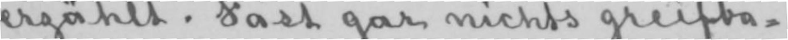erzählt. Fast gar nichts greifba-
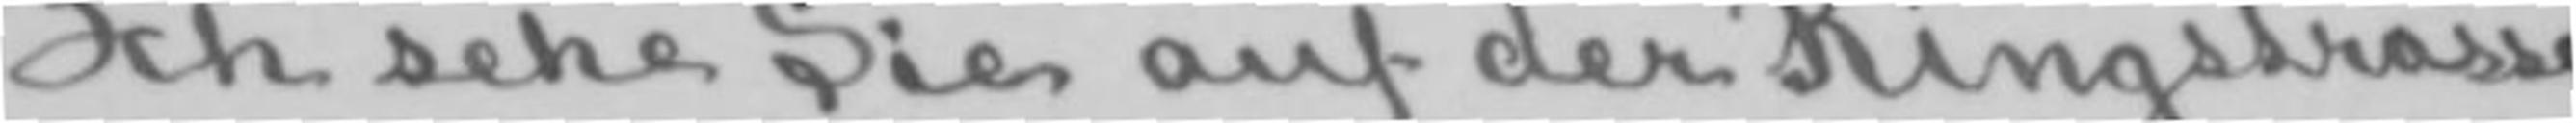Ich sehe Sie auf der Ringstrasse,
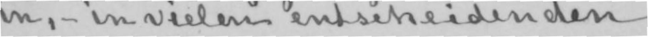in,- in vielen entscheidenden
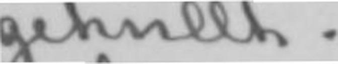gehüllt.
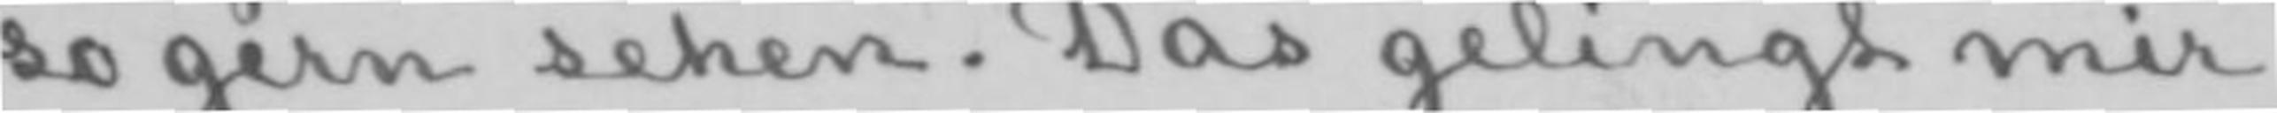so gern sehen. Das gelingt mir
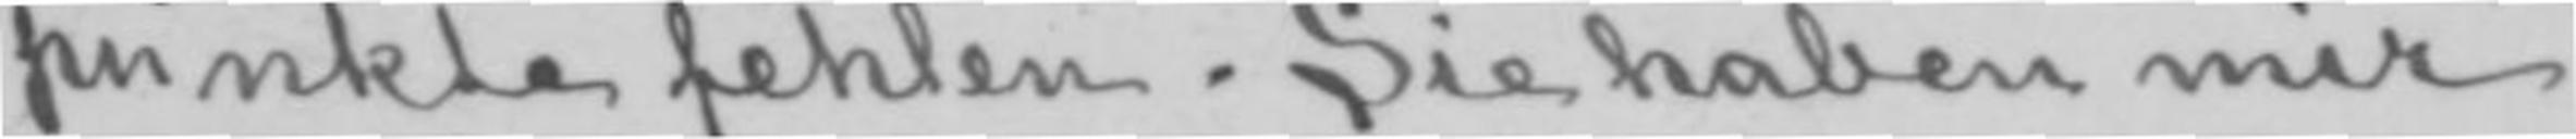pünkte fehlen. Sie haben mir
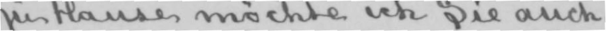Zu Hause möchte ich Sie auch
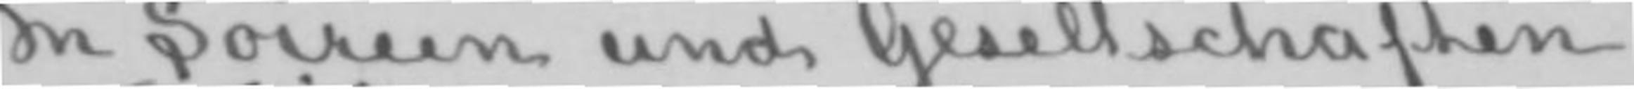In Soireen und Gesellschaften
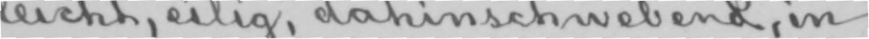leicht, eilig, dahinschwebend, in
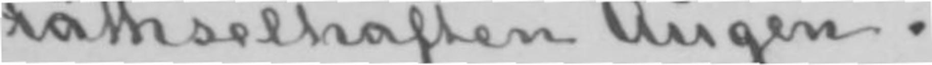räthselhaften Augen.
